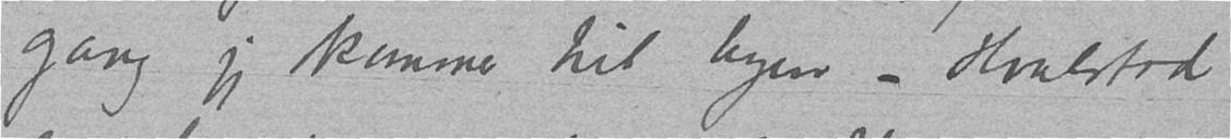gang jeg kommer til byen – Hvalstad
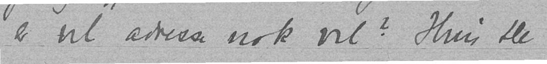er vel adresse nok vel? Hvis De
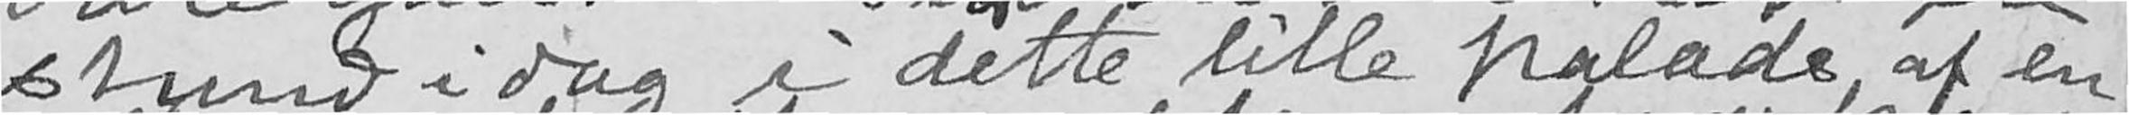stund i dag i dette lille palads af en
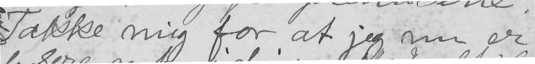Takke mig for at jeg nu er
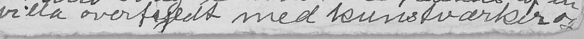villa overfyldt med kunstværker og
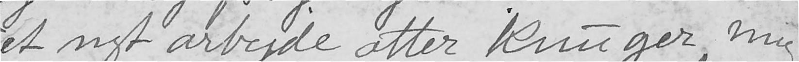et nyt arbejde atter knuger mig
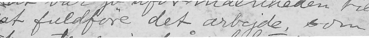at fuldføre det arbejde, som
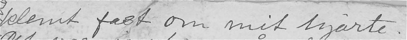klemt fast om mit hjærte.
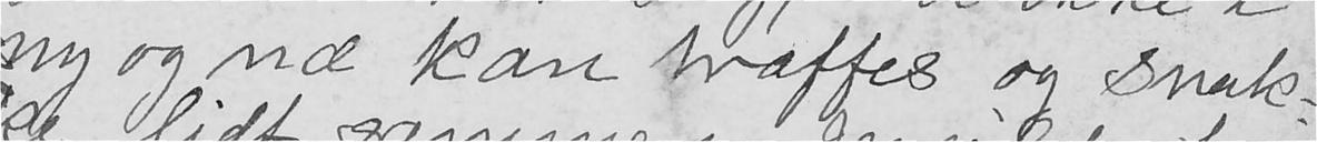ny og næ kan træffes og snak-
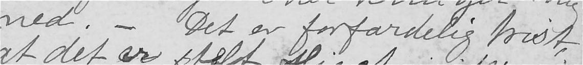ned. - Det er forfærdelig trist,
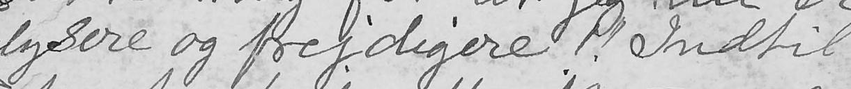lysere og frejdigere! Indtil
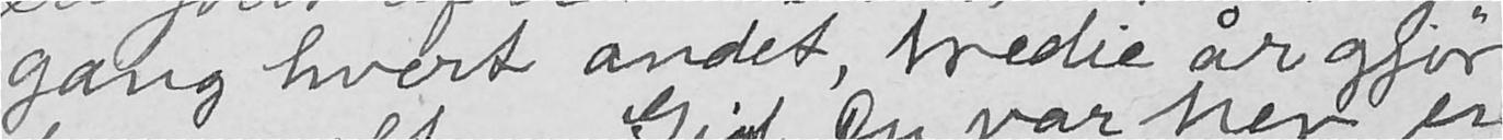gang hvert andet, tredie år gjør
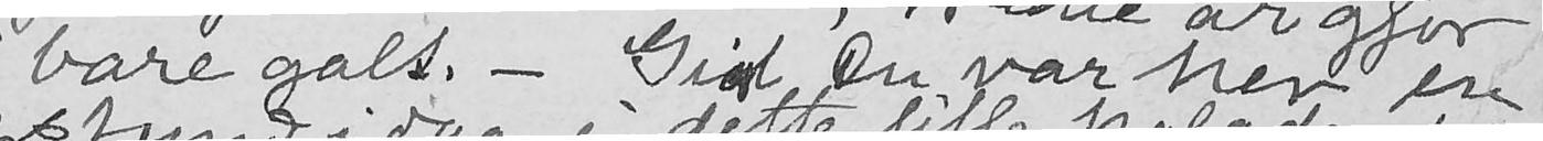bare galt. - Gid Du var her en
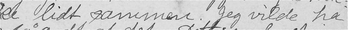ke lidt sammen. Jeg vilde ha
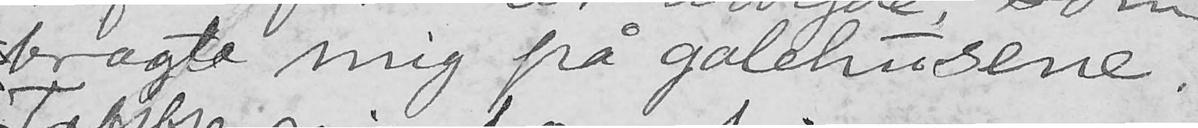bragte mig på galehusene.
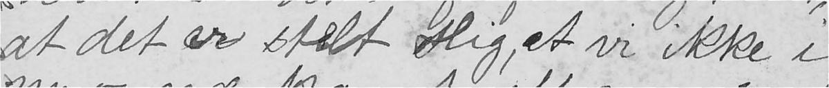at det er stelt slig, at vi ikke i
